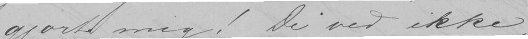gjort mig! De vedt ikke
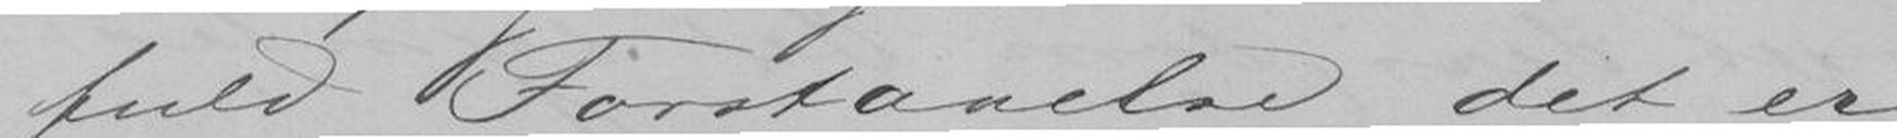fuld Forstaaelse, det er
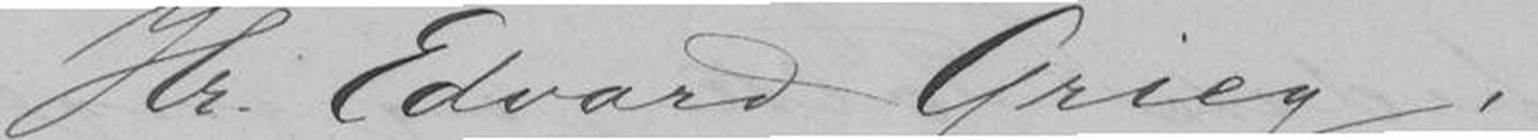Hr. Edvard Grieg!
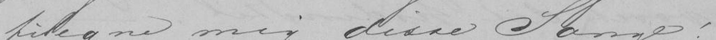tilegne mig disse Sange!
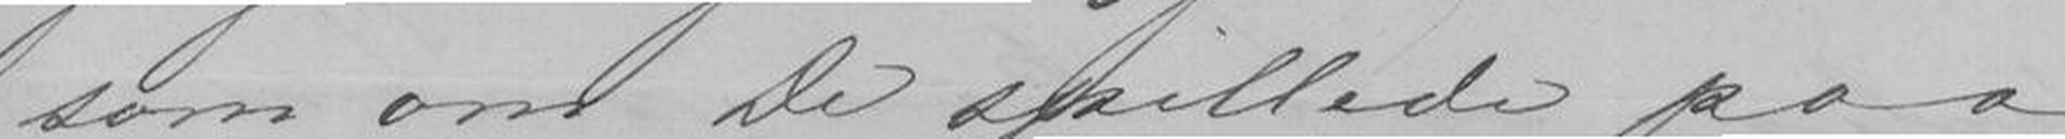som om De spillede paa
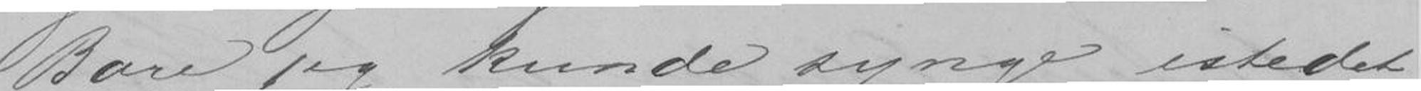Bare jeg kunde synge istedet
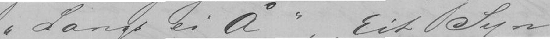Langs ei Å, Eit Syn
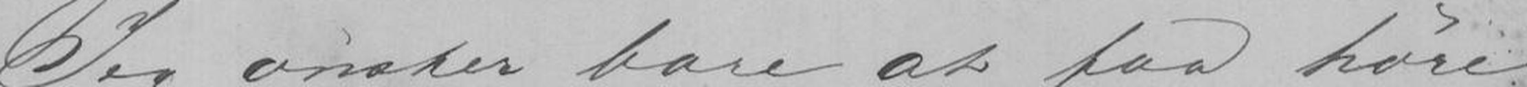Jeg ønsker bare at faa høre
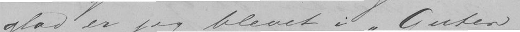glad er jeg blevet i "Guten
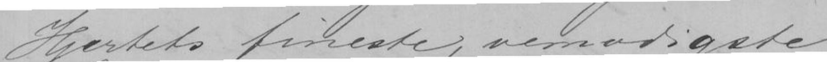Hjertets fineste, vemodigste
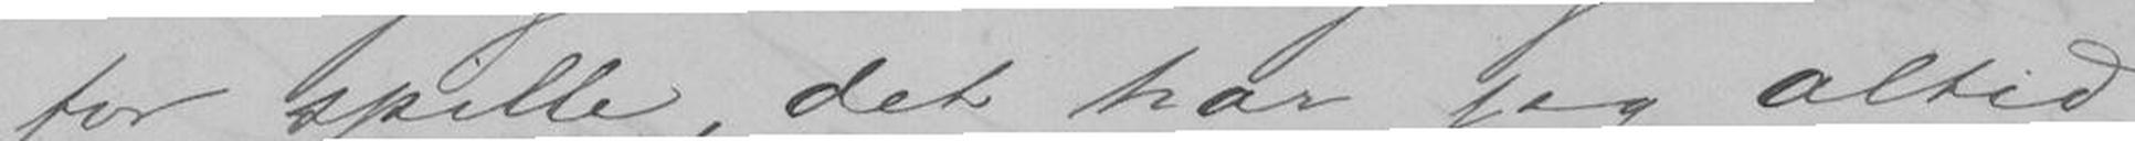for spille, det har jeg altid
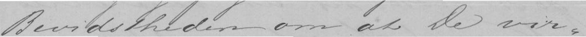Bevidstheden om at De vir-
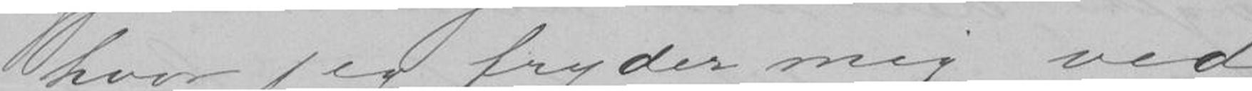hvor jeg fryder mig ved
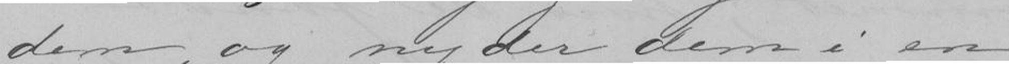dem, og nyder dem i en
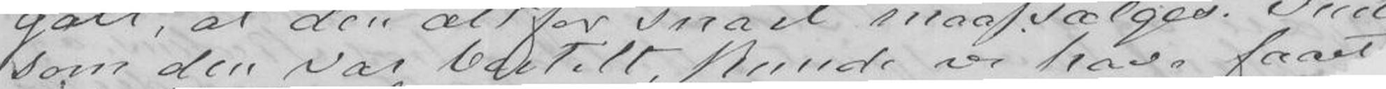som den var bestilt, kunde vi have faaet
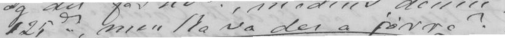125 do, men ka va der a jørre?
